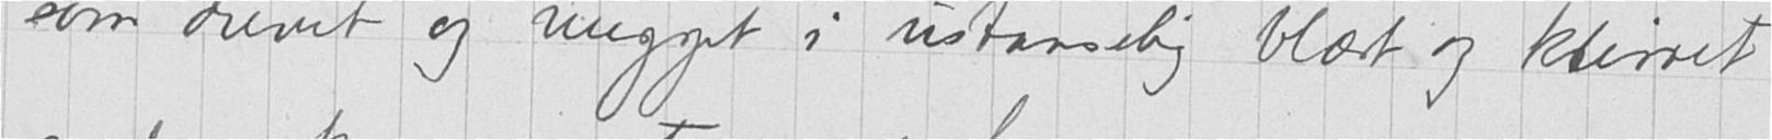som duvet og vugget i ustanselig blæst og klirret
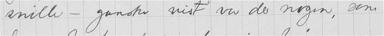snille – ganske vist var der nogen, som
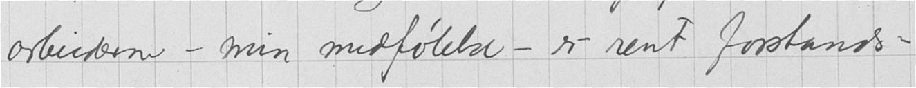arbeiderne – min medfølelse - er rent forstands-
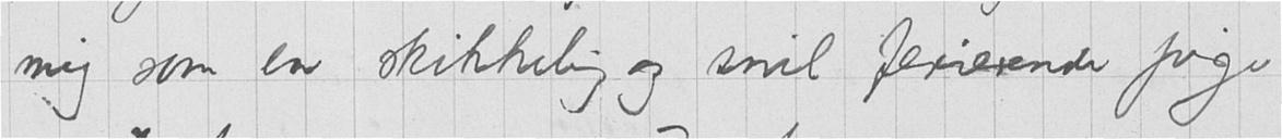mig som en skikkelig og snil ferierende pige.
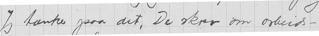Jeg tænker paa det, De skrev om arbeids-
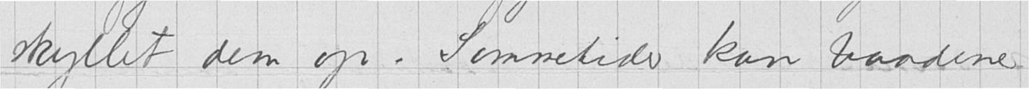skyllet dem op. Sommetider kan baaderne
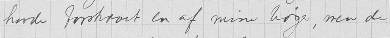havde forskrevet en af mine bøger, men de
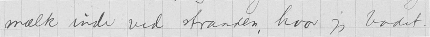mælk inde ved stranden, hvor jeg badet.
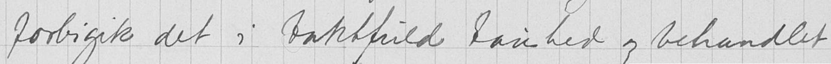forbigik det i taktfuld taushed og behandlet
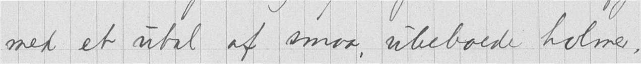med et utal af smaa, ubeboede holmer,
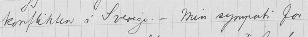konflikten i Sverige. - Min sympati for
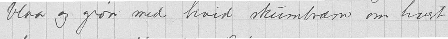blaa og graa med hvid skumbræm om hvert
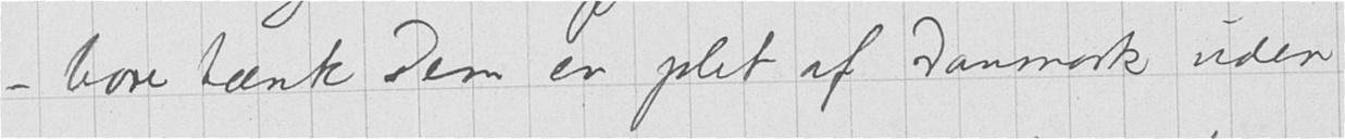– bare tænk dem en plet af Danmark uden
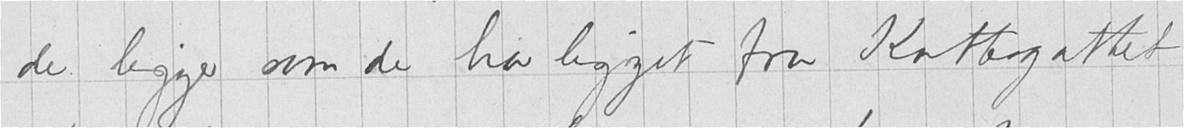de ligger som de har ligget fra Kattegattet
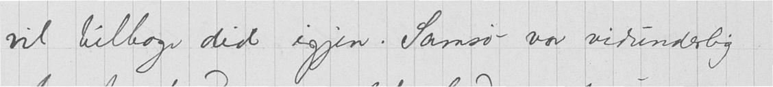vil tilbage dit igjen. Samsø  var vidunderlig
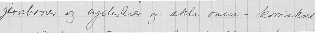jernbaner og cyclestier og ækle aviser – kornakrer
